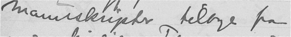manuskripter tilbage fra
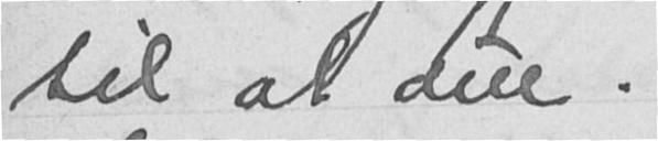til at due.
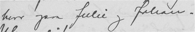hvor ogsaa Julie og Johan.
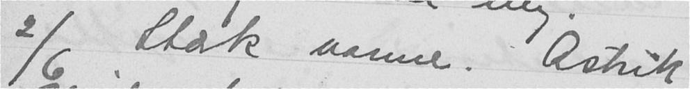2/6 Stærk varme. Astrik
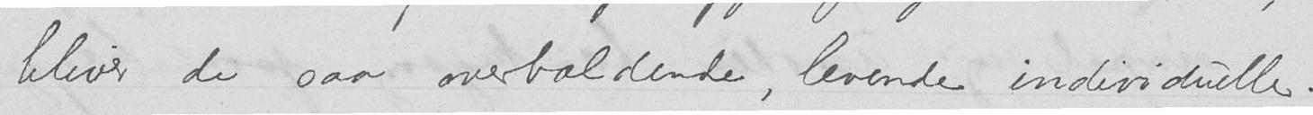bliver de saa overvældende, levende individuelle.
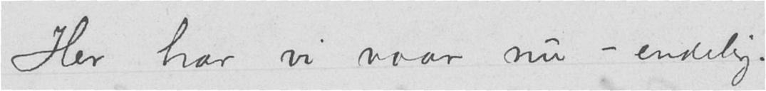Her har vi vaar nu - endelig.
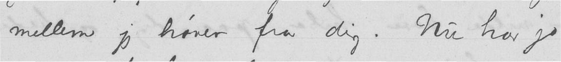mellem jeg hører fra dig. Nu har jo
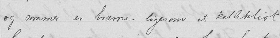og sommer er trærne ligesom et kollektivt
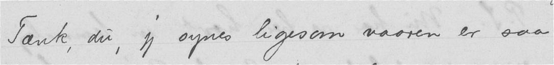Tænk, du, jeg synes ligesom vaaren er saa
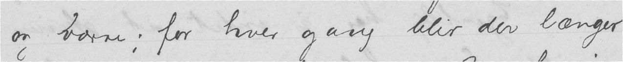og værre; for hver gang blir der længer
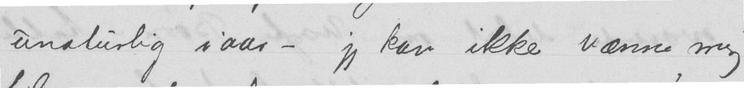unaturlig i aar - jeg kan ikke vænne mig
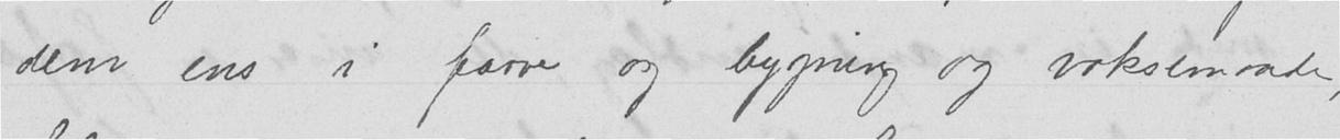dem ens i farve og bygning og voksemaade,
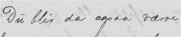Du blir da ogsaa værre
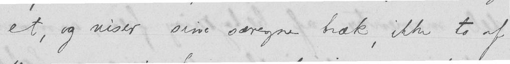et, og viser sine særegne træk, ikke to af
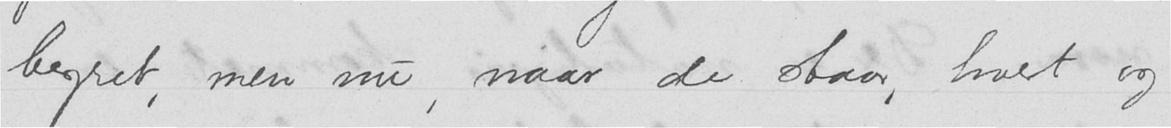begreb, men nu, naar de staar, hvert og
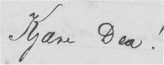Kjære Dea!
